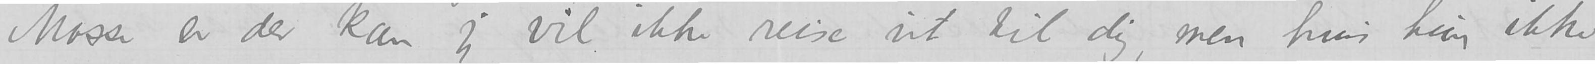Mosse er der kan jeg vil ikke reise ut til dig, men hvis hun ikke
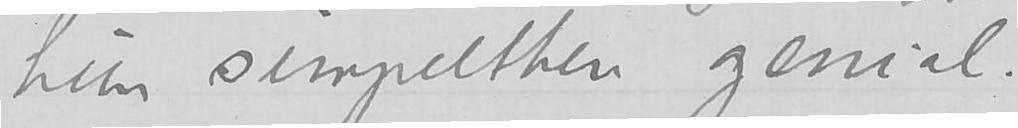hun simpelthen genial.
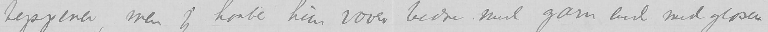teppene, men jeg haaber hun væver bedre med garn end med gloser.
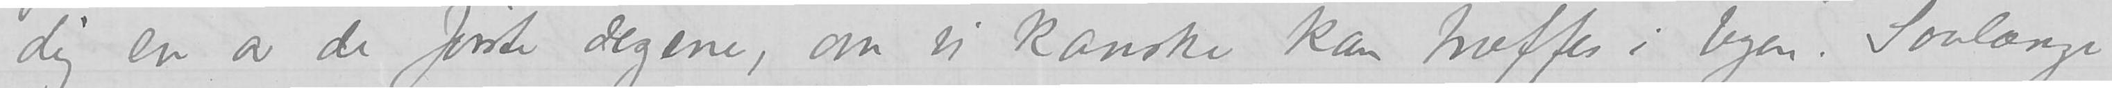dig en av de første dagene, om vi kanske kan treffes i byen. Saalænge
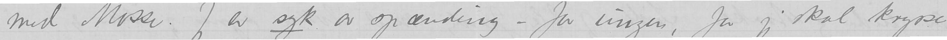med Mosse. Jeg er syk av spænding – for ungen, ja jeg skal krype
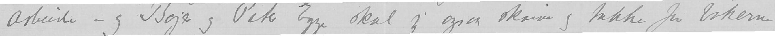arbeide – og Bojer og Peter Egge skal jeg ogsaa skrive og takke for bøkerne
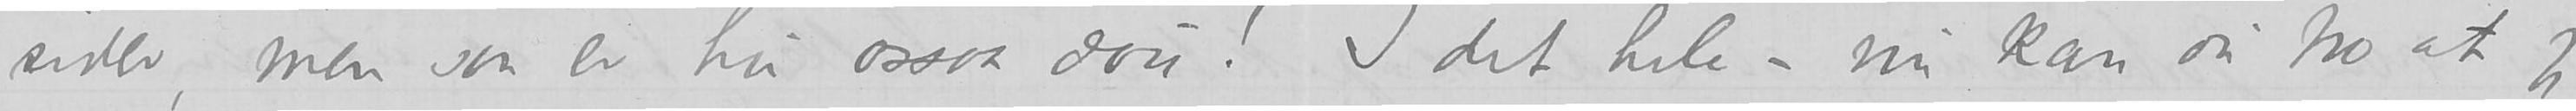sider, men saa er hu ogsaa dau! I det hele – nu kan du tro at jeg
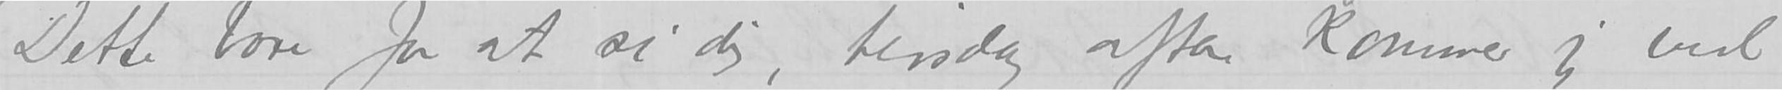Dette bare for at si dig, tirsdag aften kommer jeg ind
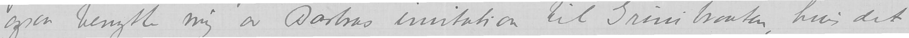ogsaa benytte mig av Barbras invitation til Grinibraaten, hvis det
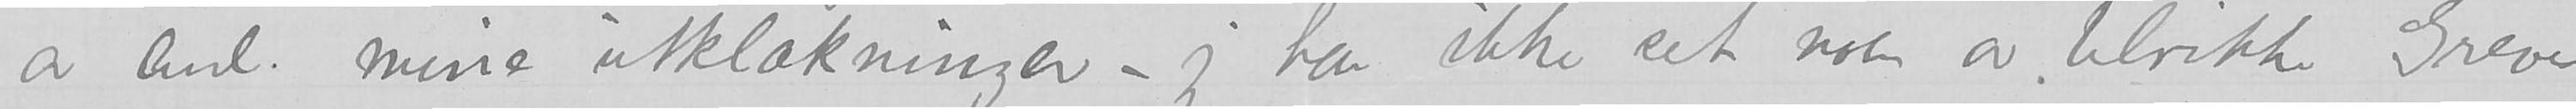av end mine utklækninger – jeg har ikke set noen av Ulrikke Greve
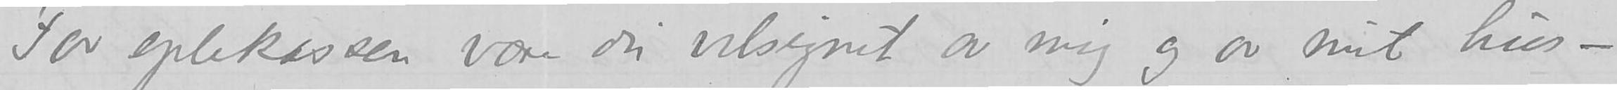For eplekassen være du velsignet av mig og av mit hus –
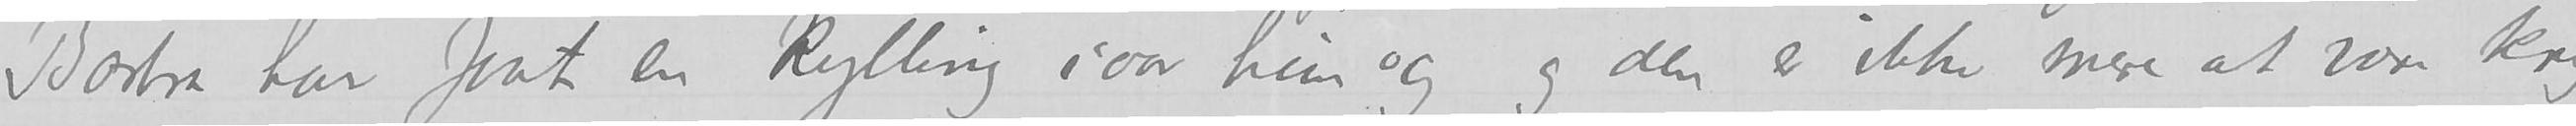Barbra har faat en kylling i aar hun og og den er ikke mere at være kry
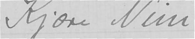Kjære Nini,
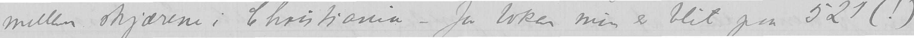mellem skjærene i Christiania – for boken min er blit paa 521 (!)
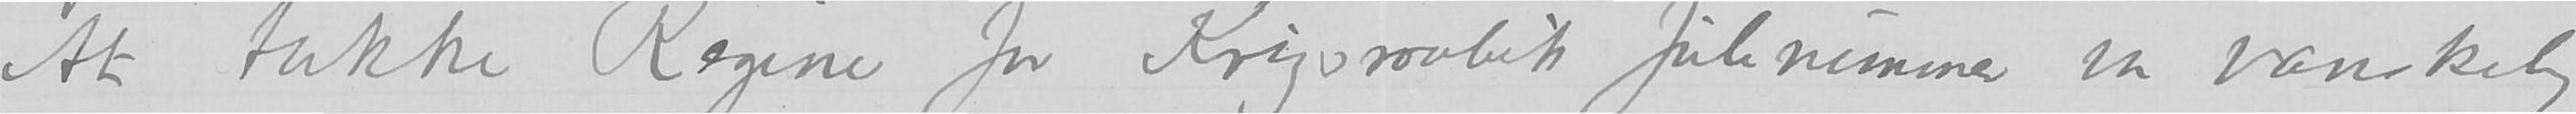At takke Regine for Krigsraabets Julenummer var vanskelig
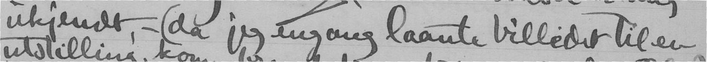ukjendt, - (da jeg engang laante billidet til en
